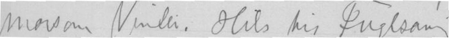morsom Vinter. Hils til Fuglsang!
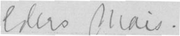Eders Mais.
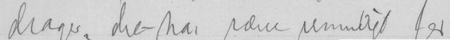I drager, det har været umuligt for
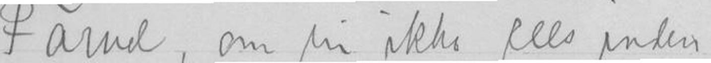Farvel, om vi ikke sees inden
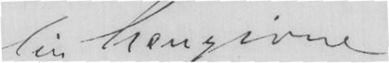Din hengivne
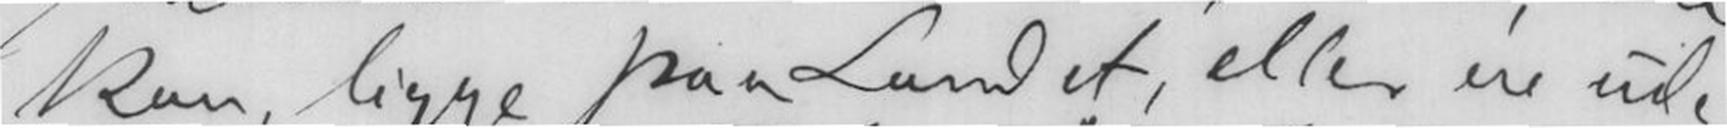kan, ligge paa Landet, eller ere ude
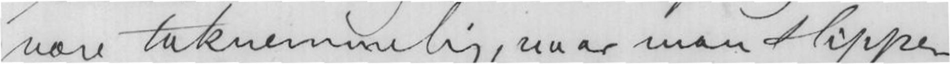være taknemmelig, naar man slipper
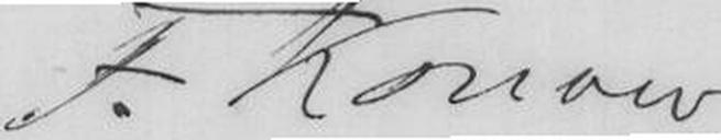F. Konow.
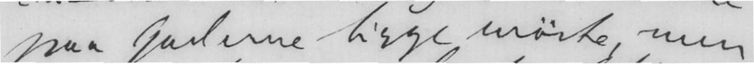paa Gaderne ligge mørke, men
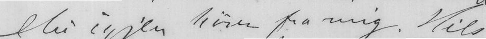Du igjen hører fra mig. Hils
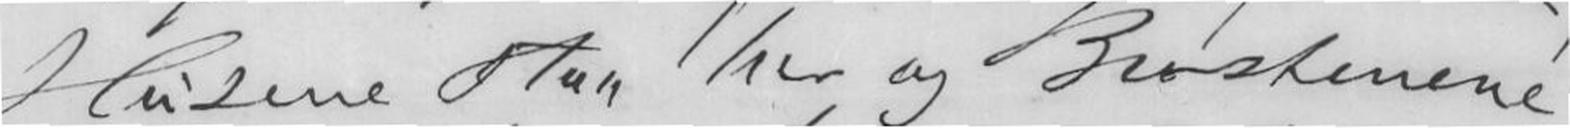Husene staa her og Brostenene
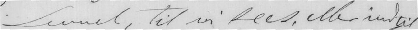Levvel, til vi sees, eller indtil
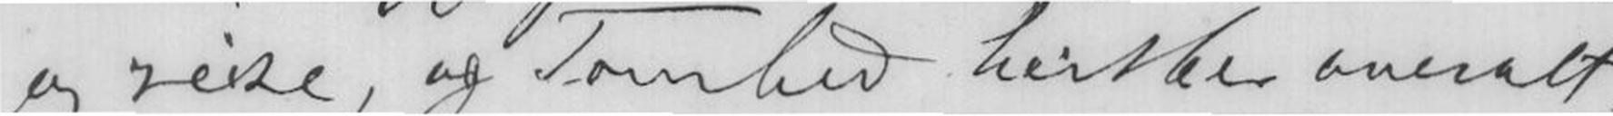og reise, og Tomhed hersker overalt.
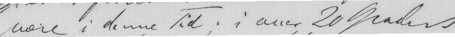være i denne Tid; i over 20 Graders
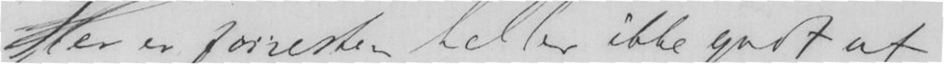Her er foresten heller ikke godt at
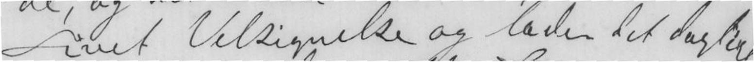Livet Velsignelse og lader det daglige
